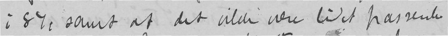i 87, samt at det vilde være lidet passende
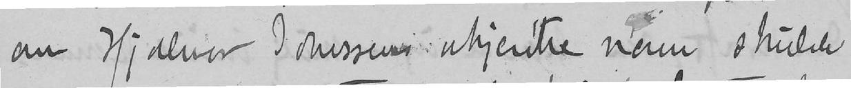om Hjalmar Johnsens ukjendte navn skulde
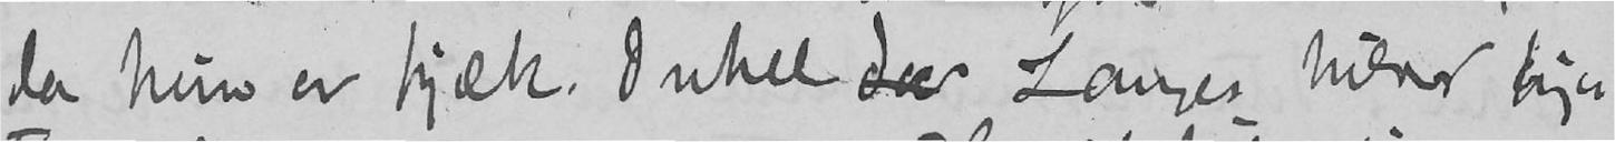da hun er kjæk. Onkel dr Lange hilser hjer
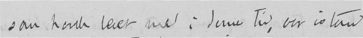som havde levet med i denne tid, var istand
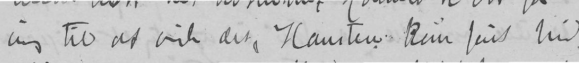ung til at vide det, Hansten kom først hid
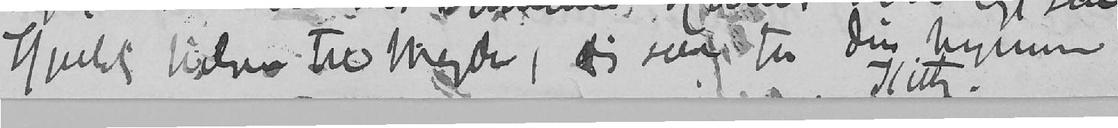Hjertelig hilsen til Magda, dig selv fra din hengivne
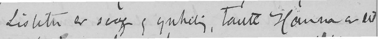Lisbeth er svag og ynkelig, tante Hanna er til
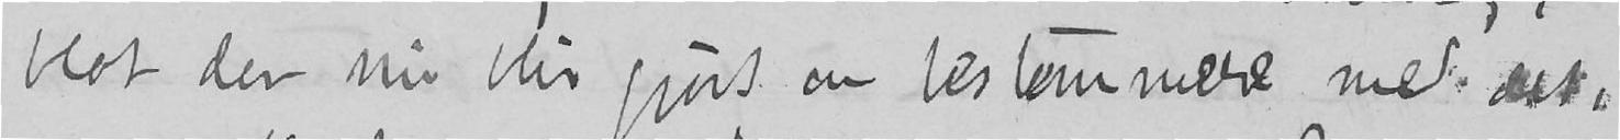blot der nu blir gjort en bestemmelse med det,
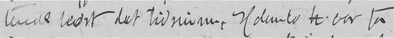terede bedst det tidsrum. Holmbo h var for
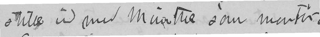stille ud med Munthe som montør.
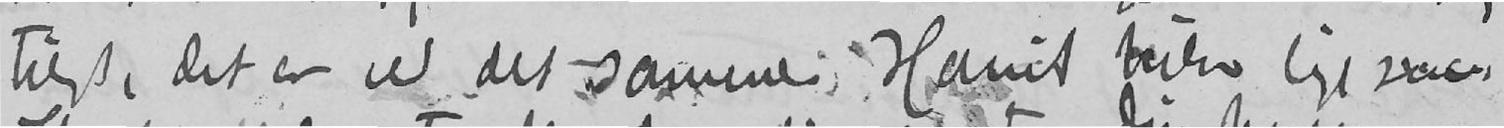teligt, det er ved det samme. Harriet hilser ligesaa.
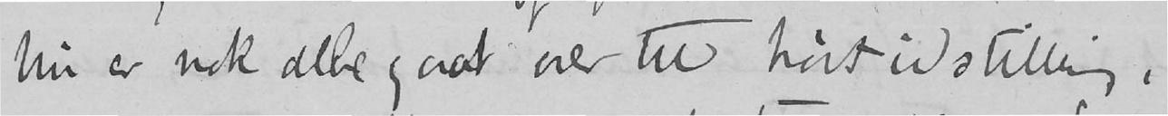Nu er nok alle gaat over til høstudstilling,
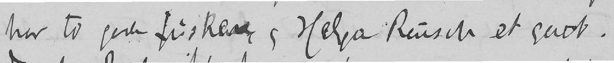har to gode friskere og Helga Reusch et godt.
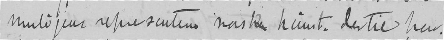muligens representere norsk kunst. Dertil hav-
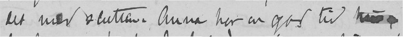det mod slutten. Anna har en god tid hu,
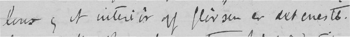lows og et interiør af Glørsen er det eneste.
